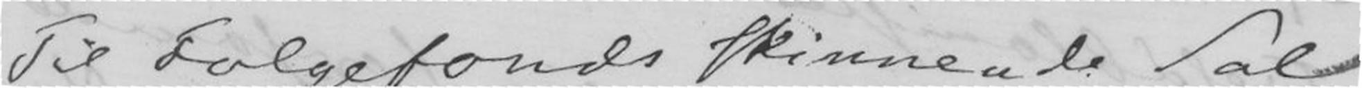Til Folgefonds Skinnende Sal.
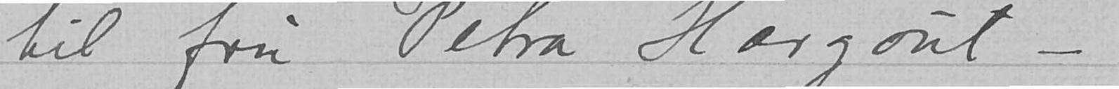til fru Petra Hærgout –
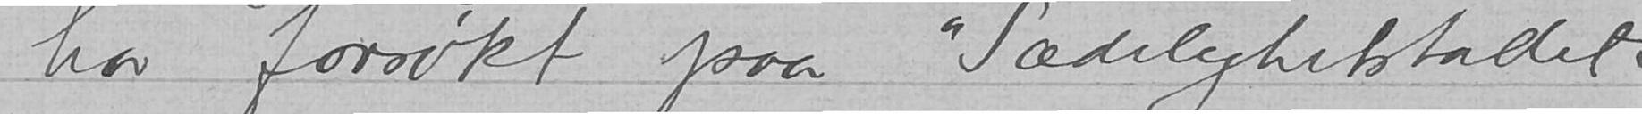Jeg har forsøkt paa «Sædelighetsballet»
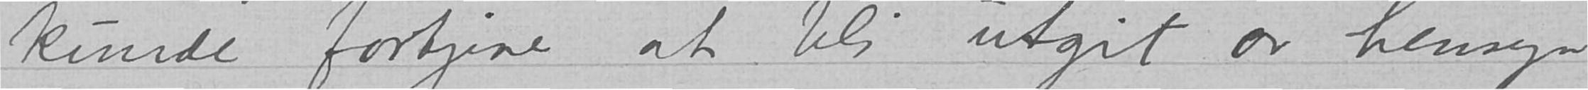J kunde fortjene at bli utgit av hensyn til fru Petra <Hærgout> –
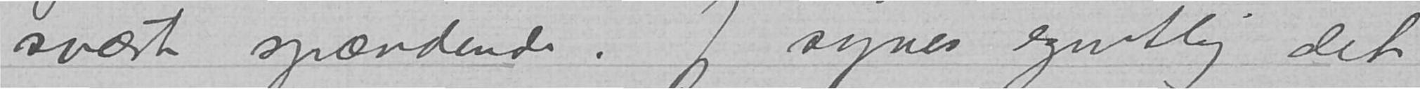svært spændende. Jeg synes egentlig det
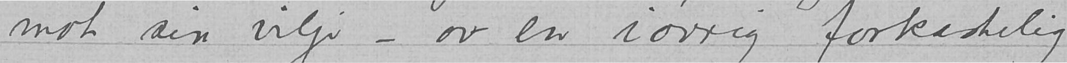mot sin vilje – av en iøvrig forkastelig.
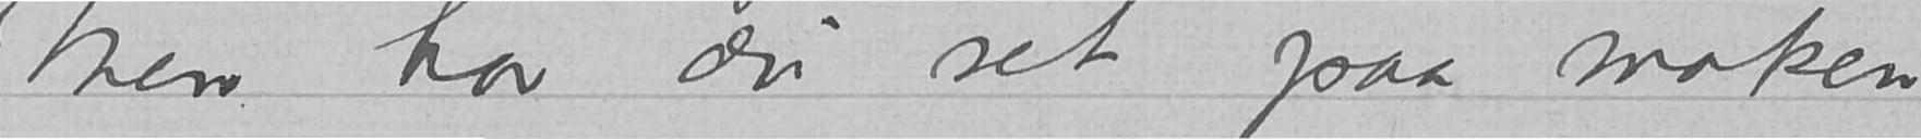Men har du set paa maken
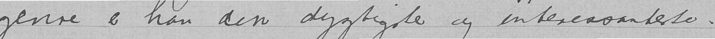genre er han den dygtigste og interessanteste.		Tak for «Firfeier» {komedie, 1912}.  svært spændende. J kunde fortjene at bli utgit av hensyn til fru Petra <Hærgout> –.
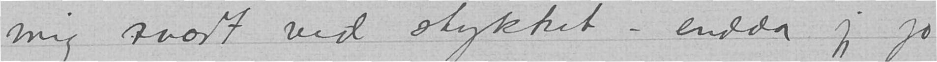mig svært ved stykket – endda jeg jo
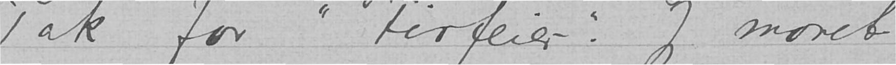Tak for «Firfeier» {komedie, 1912}. Jeg moret
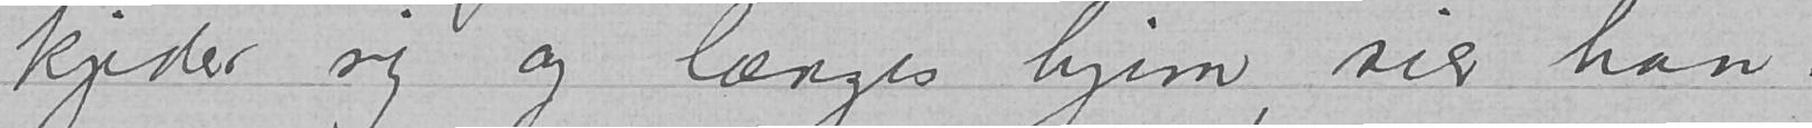kjeder sig og længes hjem, sier  han.
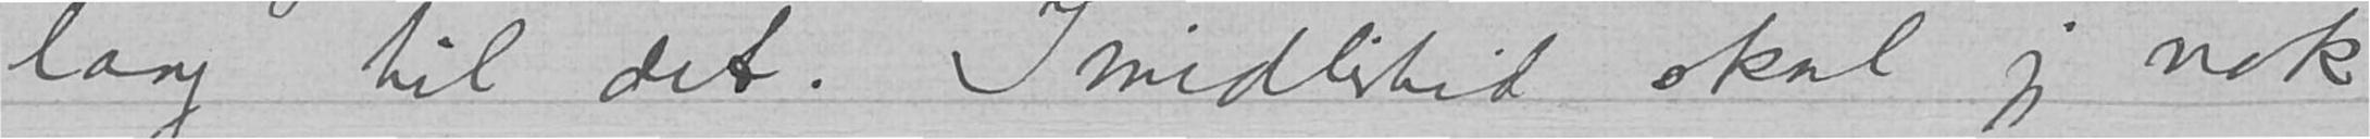lang til det. Imidlertid skal jeg nok
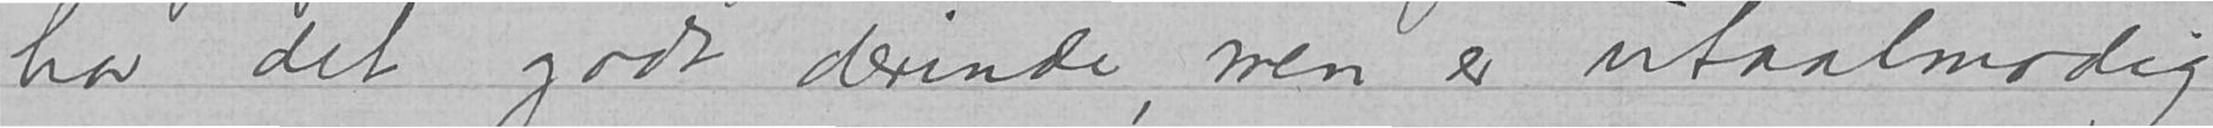har det godt derinde, men er utaalmodig,
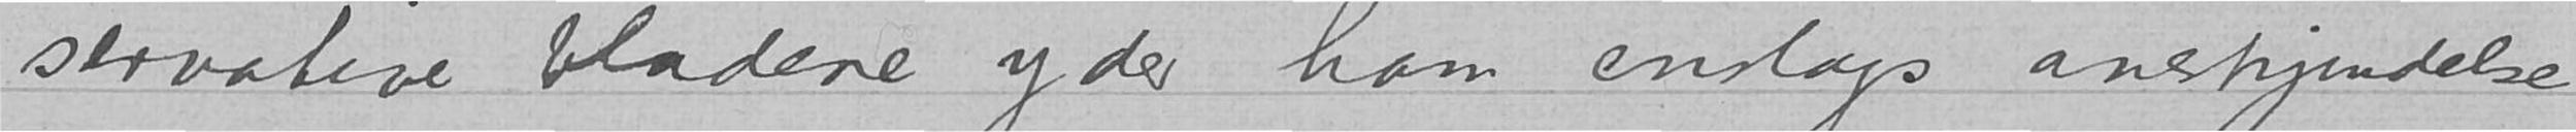servative bladene yder ham enslags anerkjendelse.
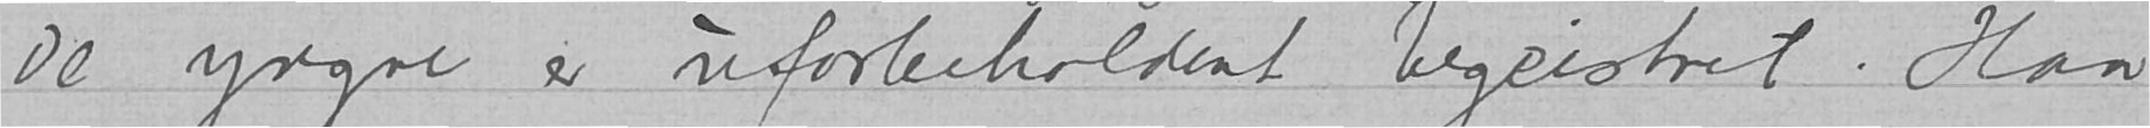De yngre er uforbeholdent begeistret Han		Men har du set paa maken
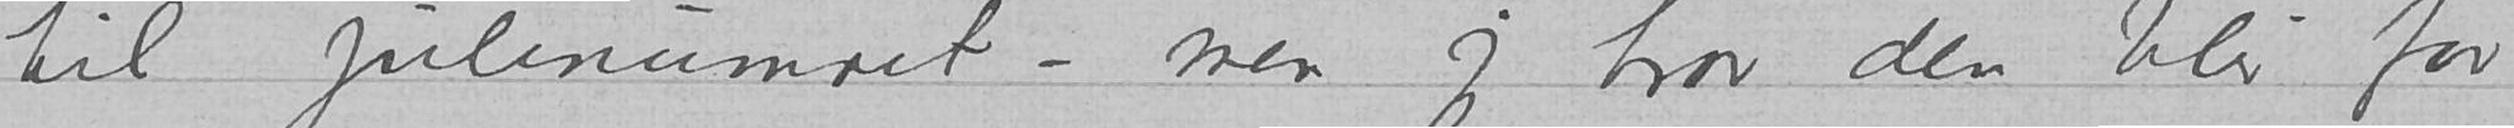til julenumret – men jeg tror den blir for
